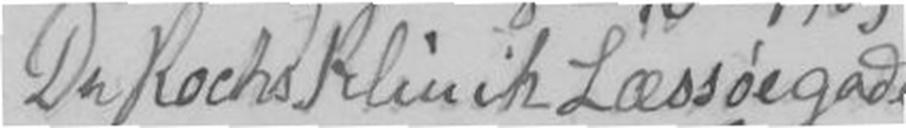Du Rochs Klinick Læssøegade
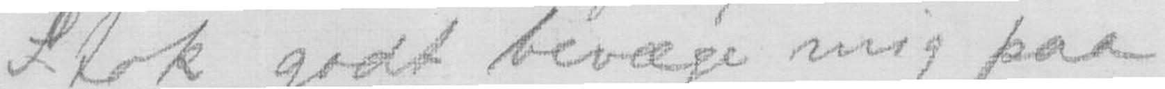Stok godt bevæge mig paa
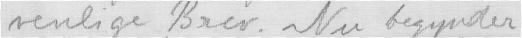venlige Brev. Nu begynder
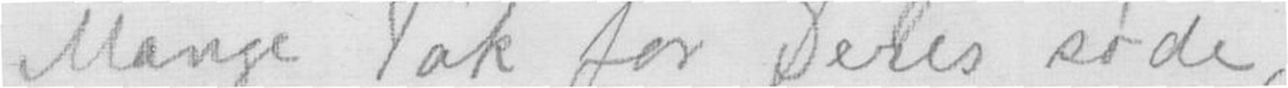Mange Tak for Deres søde,
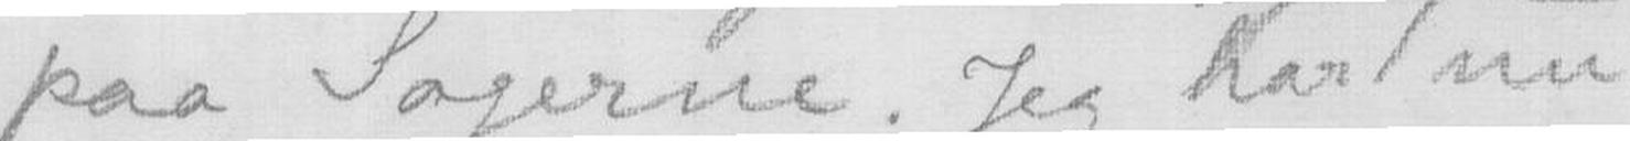paa Sagerne. Jeg har nu
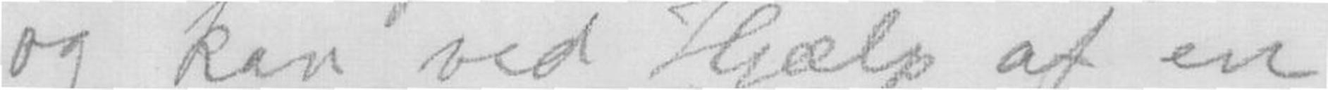og kan ved Hjælp af en
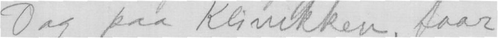Dag paa Klinikken, faar
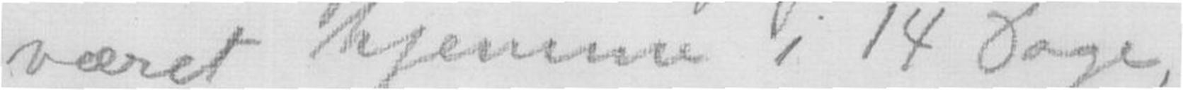været hjemme i 14 Dage,
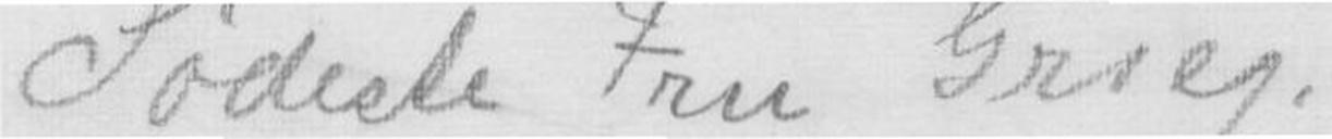Sødeste Fru Grieg.
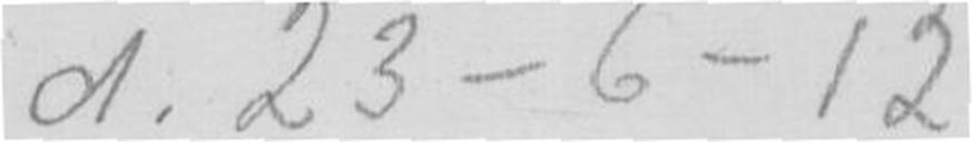d.23-6-12
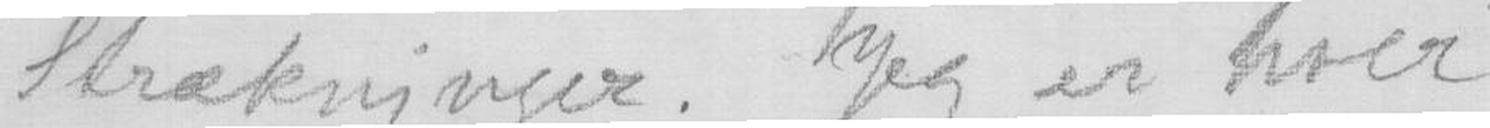Strækninger. Jeg er hver
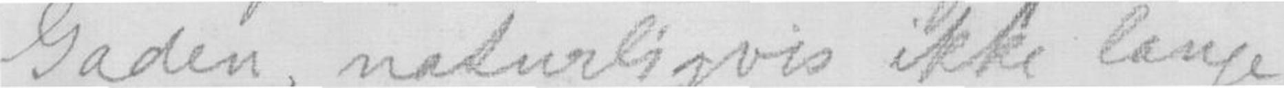Gaden, naturligvis ikke lange
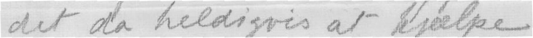det da heldigvis at hjælpe
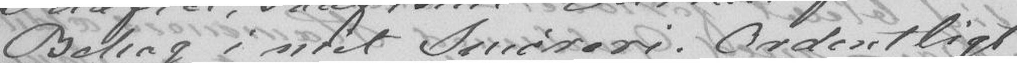Behag i mit Smøreri. Ordentligt
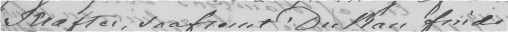Kræfter, saafremt Du kan finde
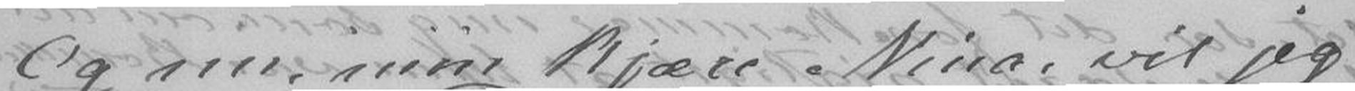Og mu min kjære Nina vil jeg
